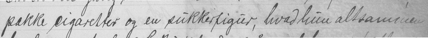pakke cigaretter og en sukkerfigur, hvad hun altsammen
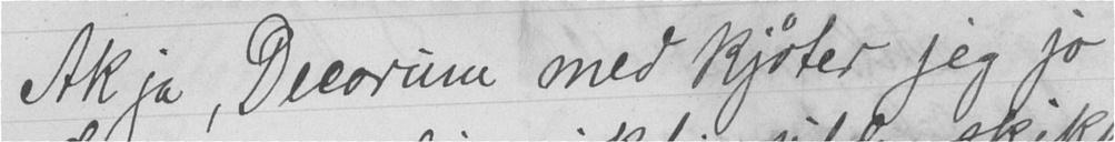Ak ja, Decorum med kjøter jeg jo
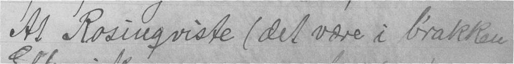At Rosinqviste (det være i b'rakken
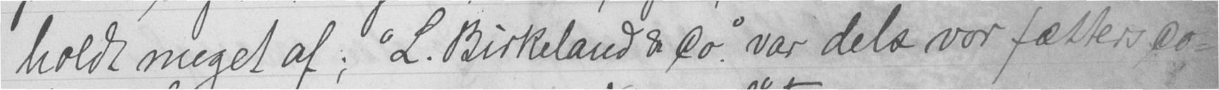holdt meget af; "L. Birkeland & co." var dels vor fætters co-
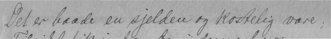Det er baade en sjælden og kostelig vare;
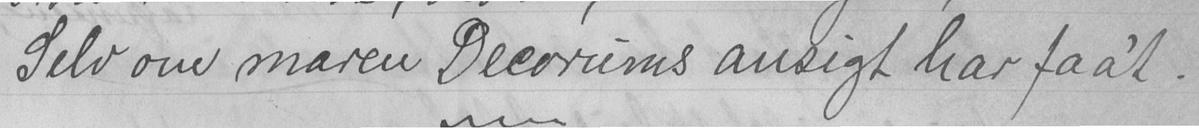Selv om maren Decorums ansigt har fa'at.
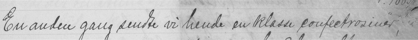En anden gang sendte vi hende en klasse confectrosiner,
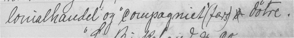lonialhandel og "compagniet " (fars) s døtre.
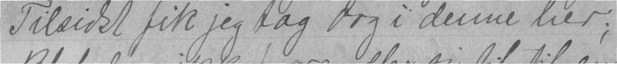Tilsidst fik jeg tag dog i denne her;
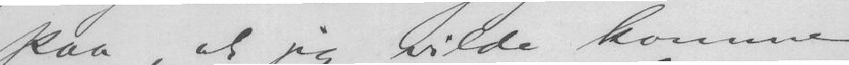paa, at jeg vilde komme
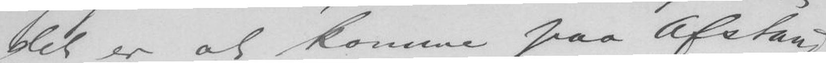det er at komme paa Afstand en-
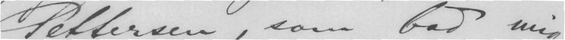Pettersen, som bad mig
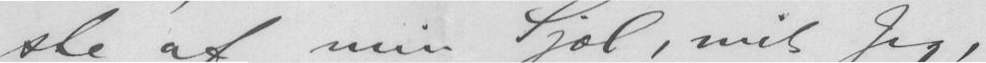ste af min Sjæl, mit Jeg,
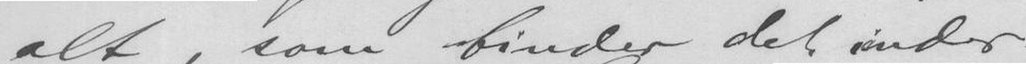alt, som binder det inder-
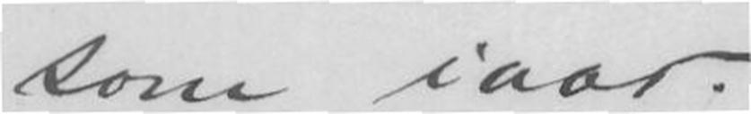som iaar.
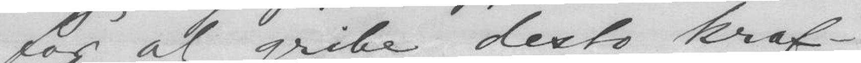for at gribe desto kraf-
